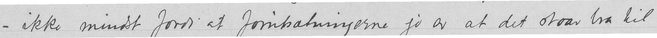– ikke mindst fordi at forutsætningerne jo er at det staar bra til
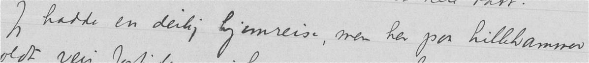Jeg hadde en deilig hjemreise, men her paa Lillehammer
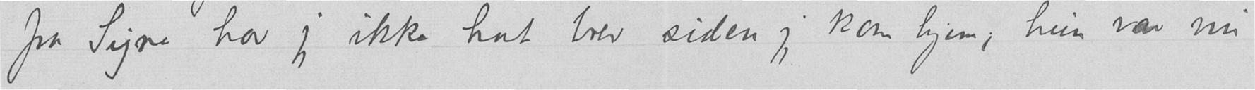fra Signe har jeg ikke hat brev siden jeg kom hjem; hun var nu
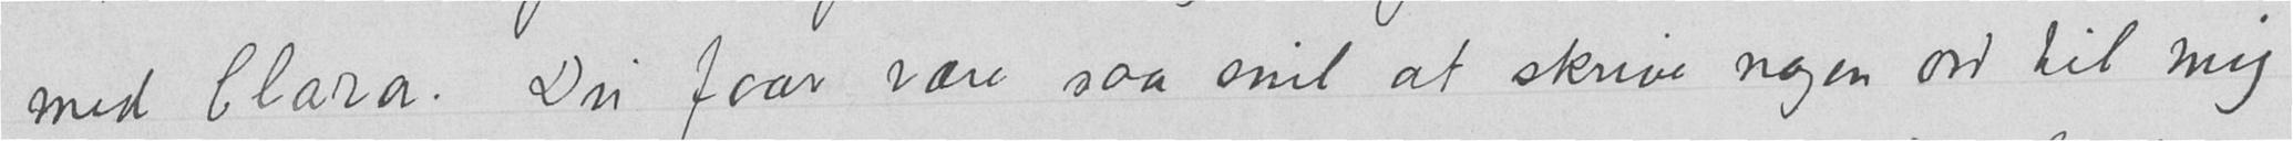med Clara. Du faar være saa snil at skrive nogen ord til mig
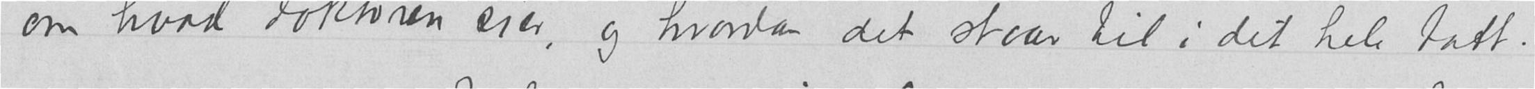om hvad doktoren sier, og hvordan det staar til i det hele tatt.
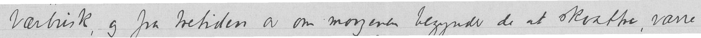bærbusk, og fra tretiden av om morgenen begynder de at skvattre, værre
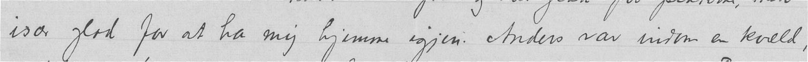især glad for at ha mig hjemme igjen. Anders var indom en kveld,
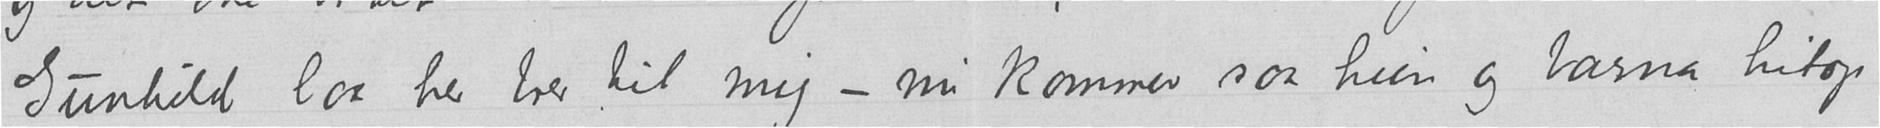Gunhild laa her brev til mig – nu kommer saa hun og barna hitop
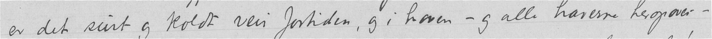er det surt og koldt veir fortiden, og i haven - og alle haverne heropover –
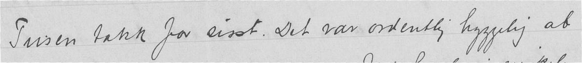Tusen takk for sisst. Det var ordentlig hyggelig at
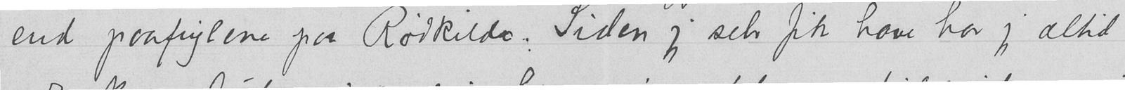end paafuglene paa Rødkilde. Siden jeg selv fik have har jeg altid
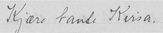Kjære tande Kirsa.
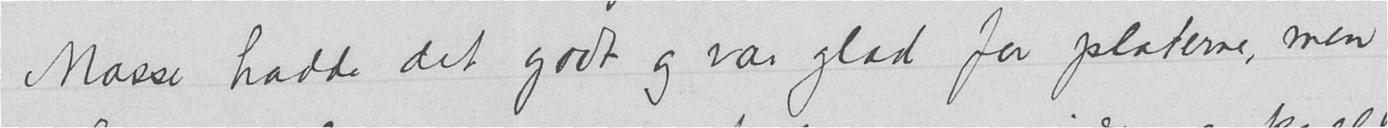Mosse hadde det godt og var glad for platerne, men
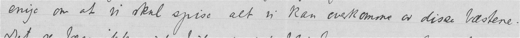enige om at vi skal spise alt vi kan overkomme av disse bæstene.
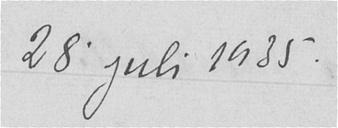28. juli 1935.
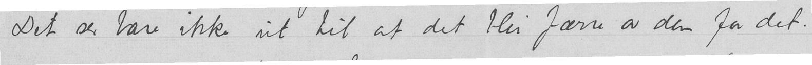Det ser bare ikke ut til at det blir færre av dem for det.
